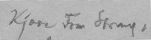Kjære Fru Stray,
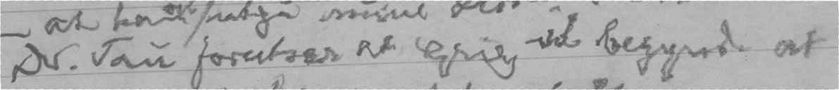Dr. Tau forutser at Grieg vil begynde at
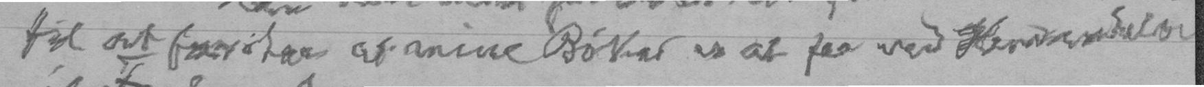til at forstaa at mine Bøker er at faa ved Henvendelse
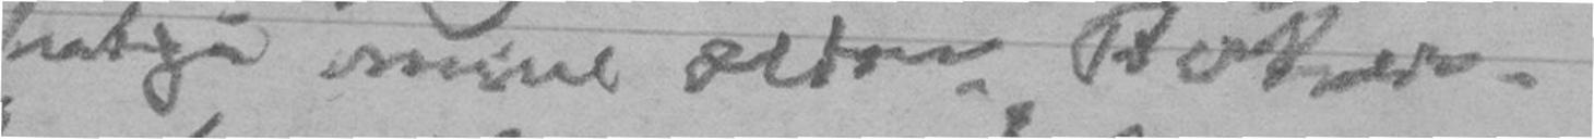utgi mine ældre Bøker.
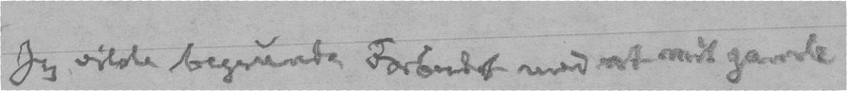Jeg vilde begrunde Forbudet med at mit gamle
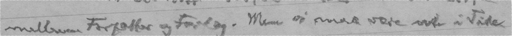mellem Forfatter og Forlag. Men vi maa være ute i Tide
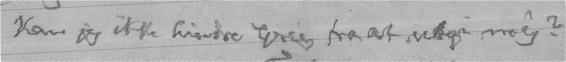Kan jeg ikke hindre Grieg fra at utgi mig?
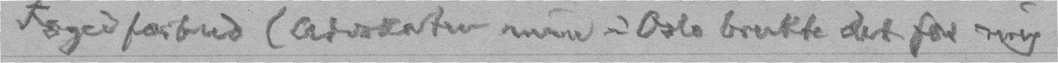Fogedforbud (Advokaten min i Oslo brukte det for mig
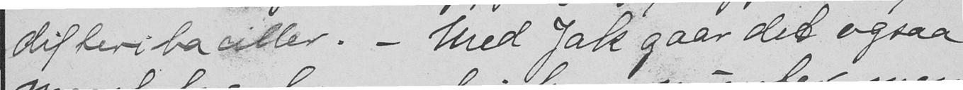difteribaciller. - Med Jak gaar det ogsaa
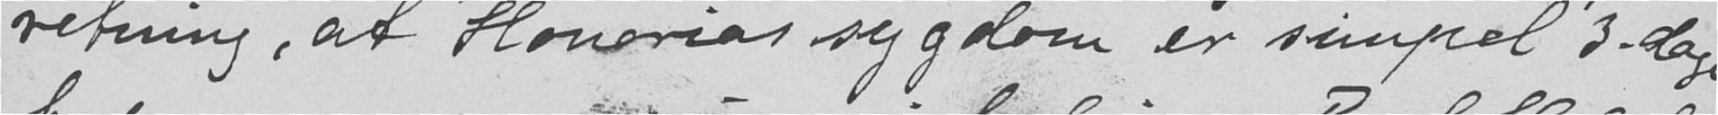retning, at Honorias sygdom er simpel 3-dagers
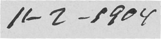11-2-1904
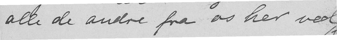alle de andre fra os her ved
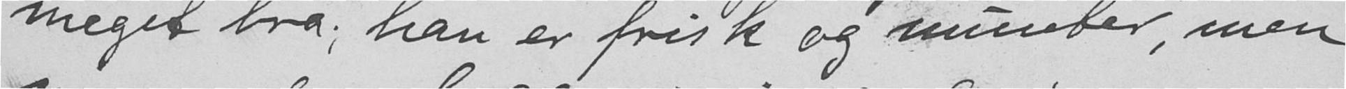meget bra; han er frisk og munter, men
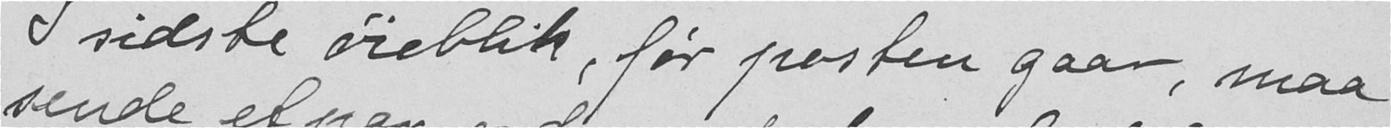I sidste øieblik, før posten gaar, maa
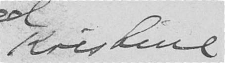Kristine
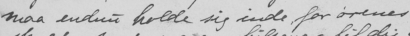maa endnu holde sig inde for ørenes
